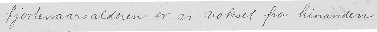fjortenaarsalderen er vi vokset fra hinanden
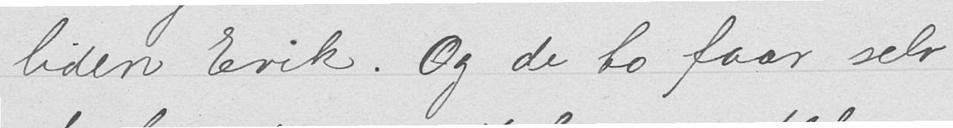liden Erik. Og de to faar selv
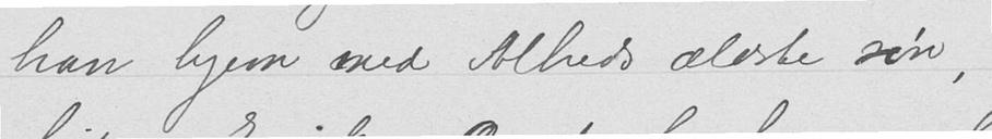han hjem med Alheds ældste søn,
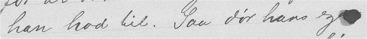han had til. Saa dør hans egen
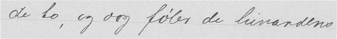de to, og dog føler de hinandens
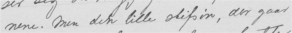nene. Men den lille stifsøn, der gaar
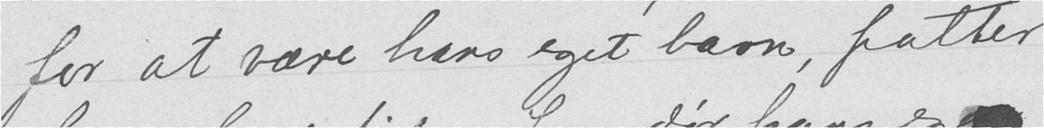for at være hans eget barn, fatter
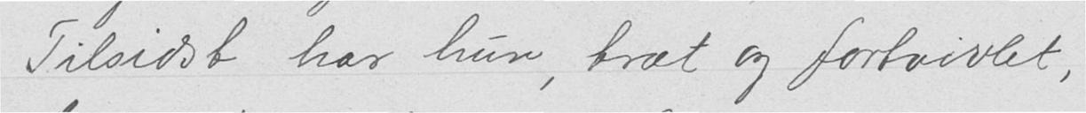Tilsidst har hun, træt og fortvivlet,
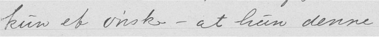kun et ønske – at hun denne
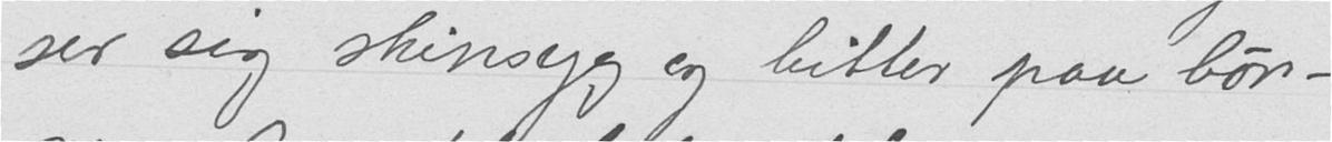ser sig skinsygg og bitter paa bør-
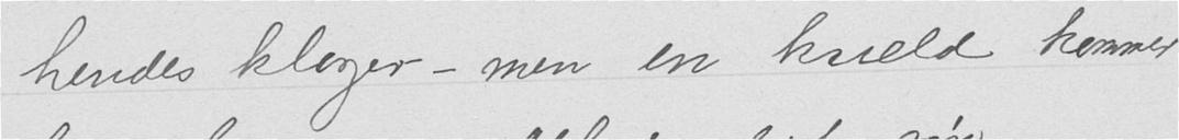hendes klager – men en kveld kommer
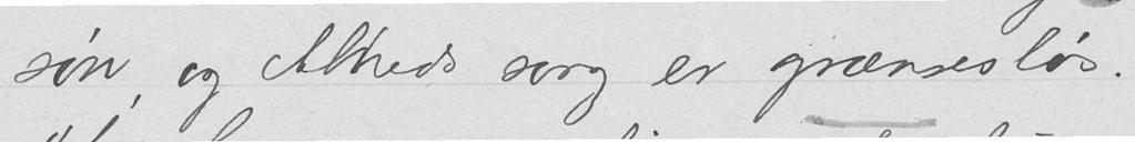søn, og Alheds sorg er grænsesløs.
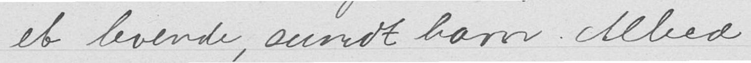et levende, sundt barn. Alhed
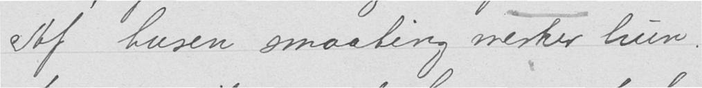Af tusen smaating merker hun,
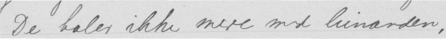De taler ikke mere med hinanden,
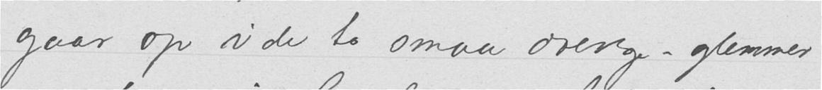gaar op i de to smaa drenge – glemmer
